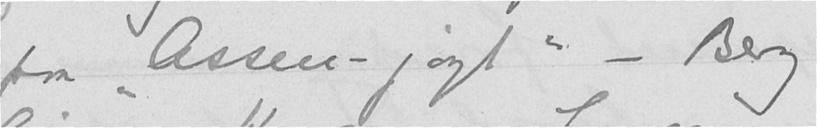paa "Assen-jagt" - Berg,
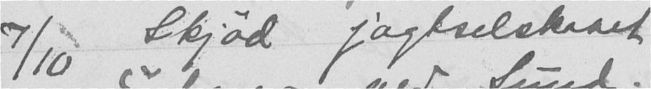7/10 Skjød jagtselskabet
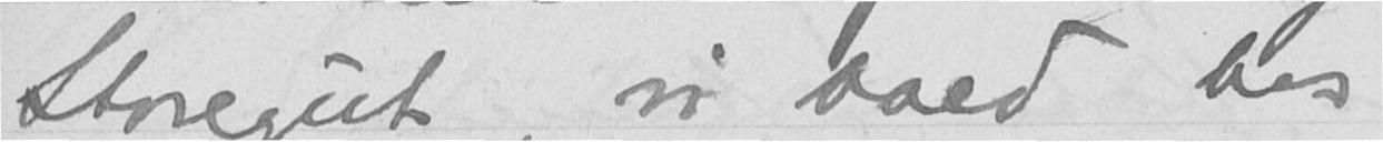Storegut, vi boed hos
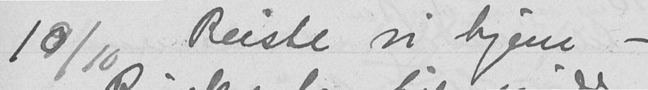10/10 Reiste vi hjem -
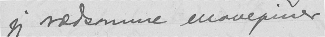jeg rædsomme mavepiner
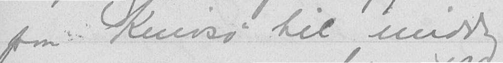paa Knivsø til middag
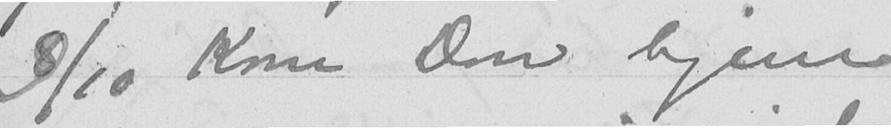9/10 Kom Don hjem
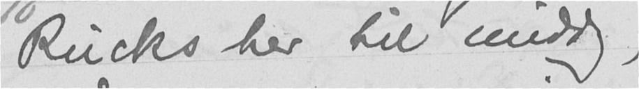Riecks her til middag,
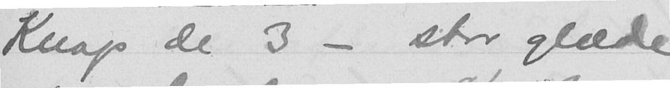Knap de 3 - stor glæde
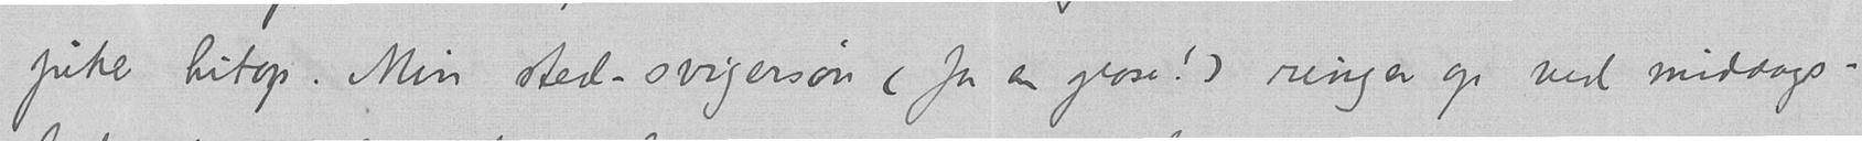pike hitop. Min sted-svigersøn (for en glose!) ringer op ved middags-
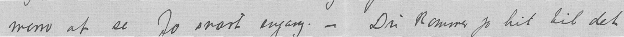morro at se Jo snart engang. – Du kommer jo hit til det
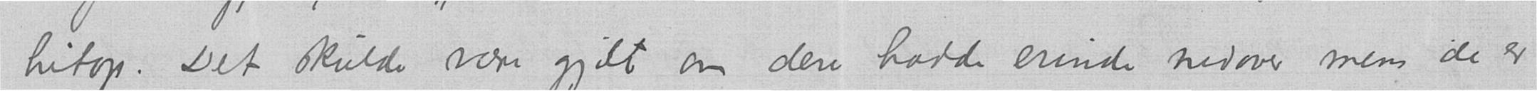hitop. Det skulde være gjilt om dere hadde erinde nedover mens de er
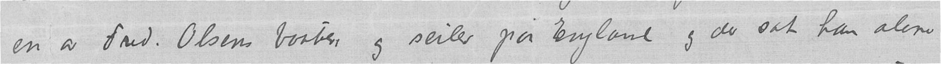en av Fred. Olsens baater og seiler paa England og der sat han alene
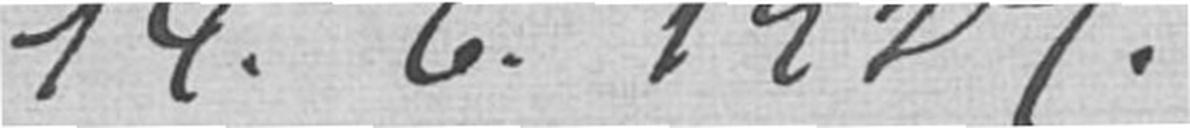14. 6. 1927.
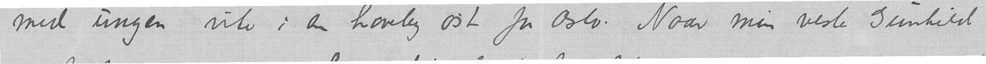med ungen ute i en haveby øst for Oslo. Naar min vesle Gunhild
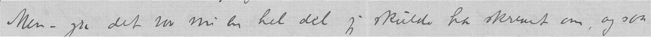Men – ja det var nu en hel del jeg skulde ha skrevet om, og saa
